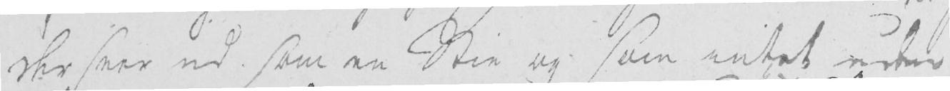der seer ud som en Stie og som intet uden
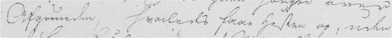Afgrunden, hvorledes faae Hesten op, uden
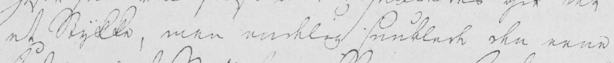et Stykke, men endelig snublede den eene
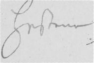Hester
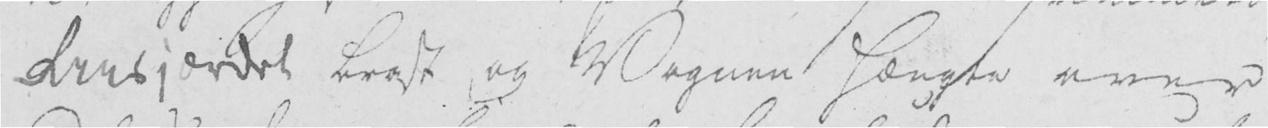Riisjordet brast og Vognen hængte over
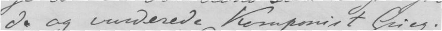de og vurderede Komponist Grieg.
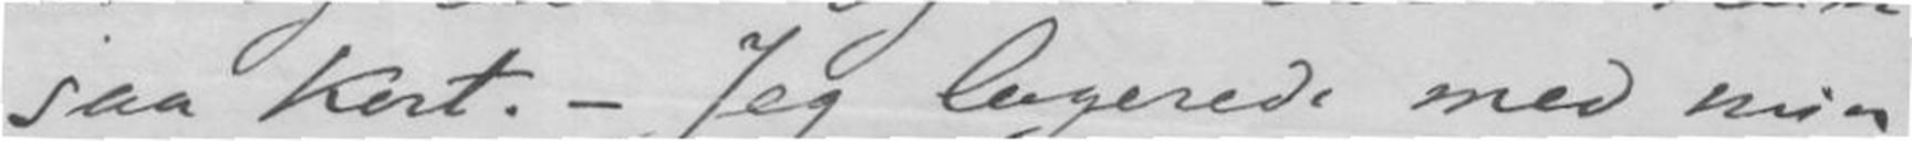saa Kort.- Jeg logerede med min
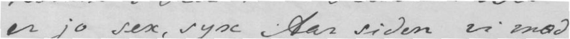er jo sex, syv Aar siden vi mød-
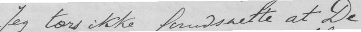Jeg tør ikke forudsette at De
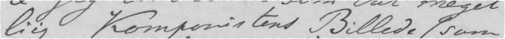liig Komponistens Billede (som
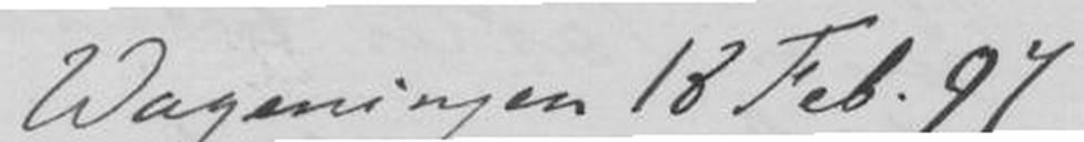Wageningen 17 Febr. 97
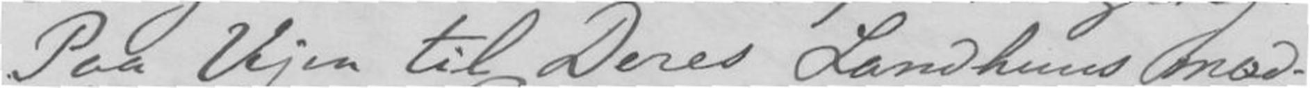Paa Vejen til Deres Landhuus mød-
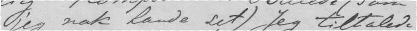jeg nok havde set) Jeg tiltalede
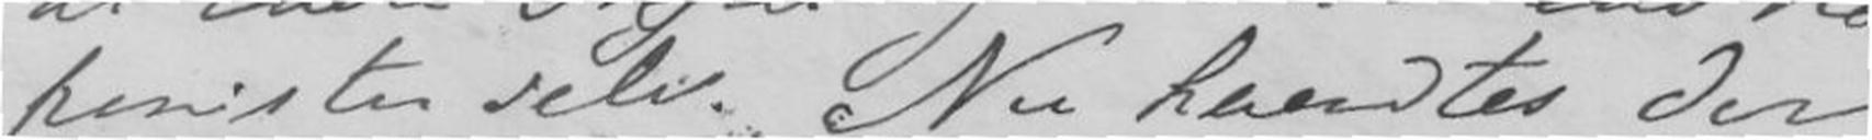ponisten selv Nu haendtes der
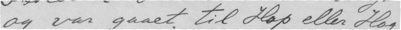og var gaaet til Hop eller Hog
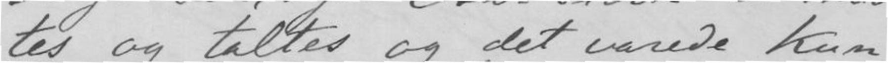tes og taltes og det varede Kun
